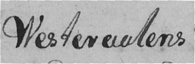Westeraalens
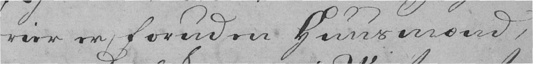rier er, foruden Huusmænd,
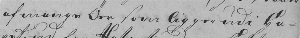af mange Øer som ligger udi Ha-
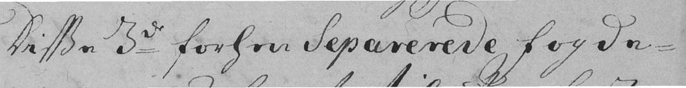Disse 3de forhen Separerede Fogde-
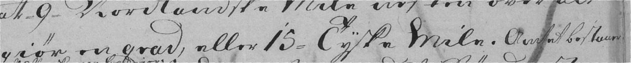giør en grad, eller 15 Tyske Mile. Amtet bestaaer
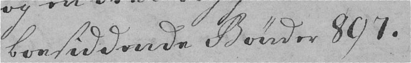boesiddende Bønder 897.
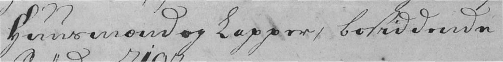Huusmænd og Lapper, bosiddende
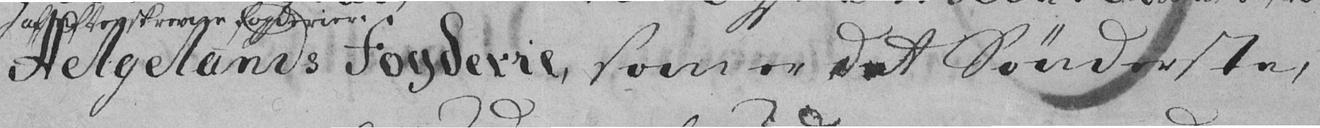Helgelands Fogderie, som er det Sønderste,
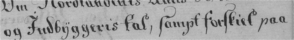og Indbyggeris Tal, sampt Forskiel paa
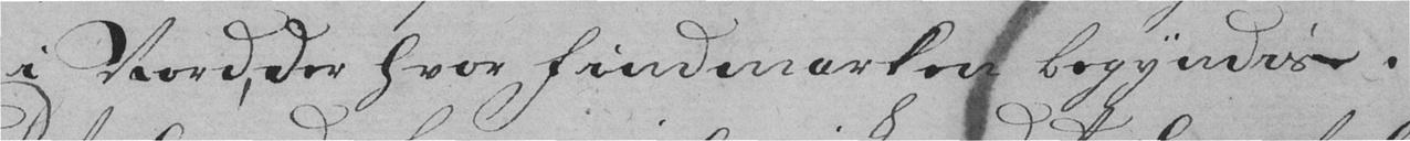i Nord, der hvor Findmarken begynder.
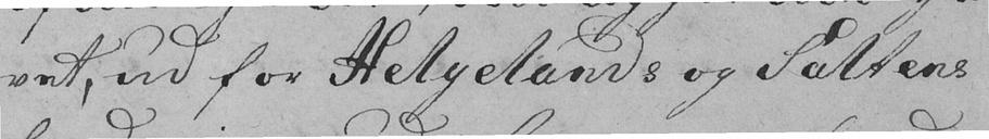vet, ud for Helgelands og Saltens
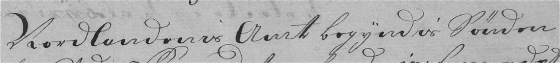Nordlandenis Amt begynder Sønden
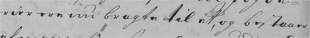rier ere nu bragte til et, og bestaaer
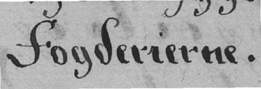Fogderierne.
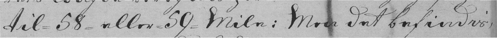til 58 eller 59 Mile: Men det befindis,
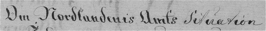Om Nordlandenis Amts Situation
